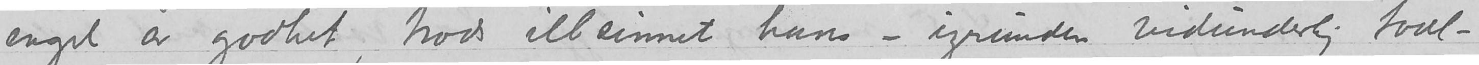engel av godhet, trods illsinnet hans – igrunden vidunderlig taal
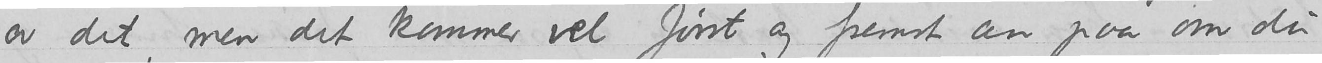av det, men det kommer vel først og fremst an paa om du
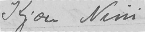Kjære Nini,
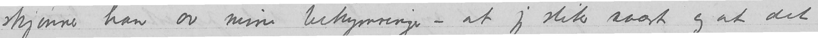skjønner han av mine bekymringer – at jeg sliter svært og at det
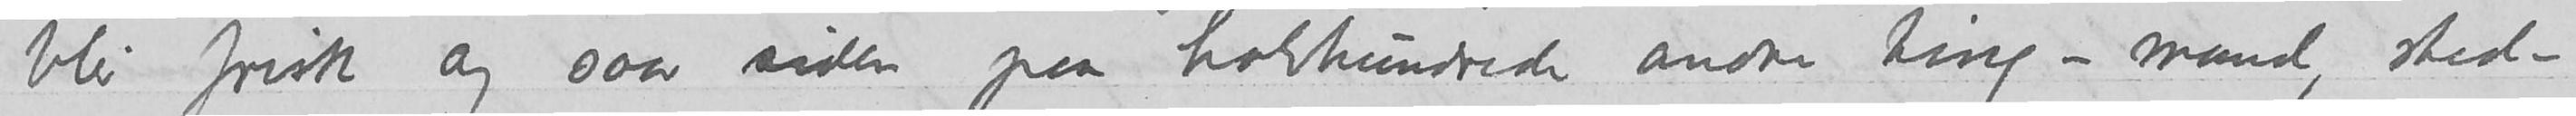blir frisk og saa siden paa halvhundrede andre ting – mand, sted-
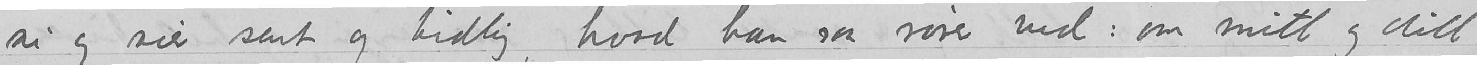si og sier sent og tidlig, hvad han saa rører ved: om mitt og ditt
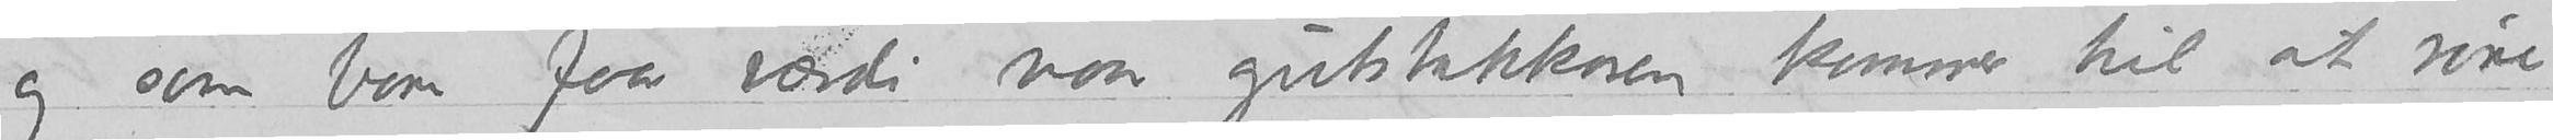og som bare faar værdi naar gutstakkaren kommer til at røre
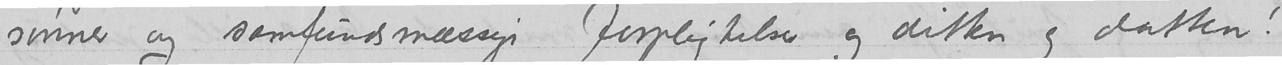sønner og samfundsmæssige forpligtelser og ditten og datten!
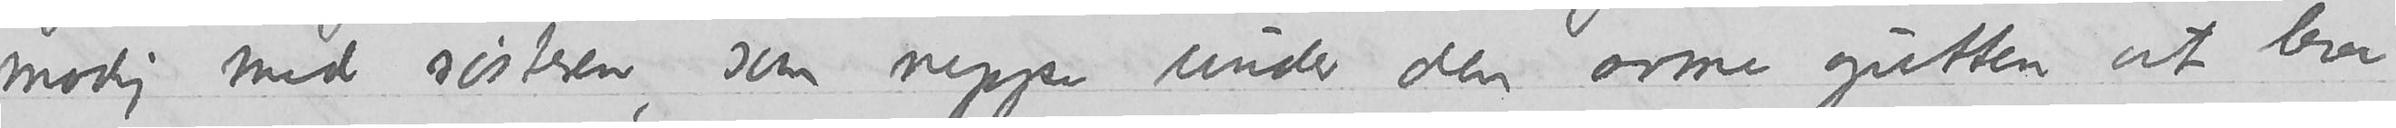modig med søsteren, som neppe under den arme gutten at leve
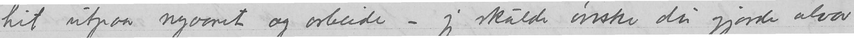hit utpaa nyaaret og arbeide – jeg skulde ønske du gjorde alvor
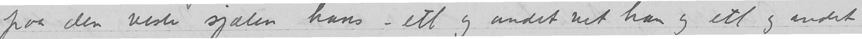paa den vesle sjælen hans – ett og andet vet han og ett og andet
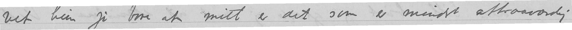vet hun jo bare at mitt er det som er mindre attraaværdig
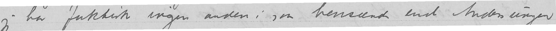jeg har faktisk ingen anden i saa henseende end Andersungen
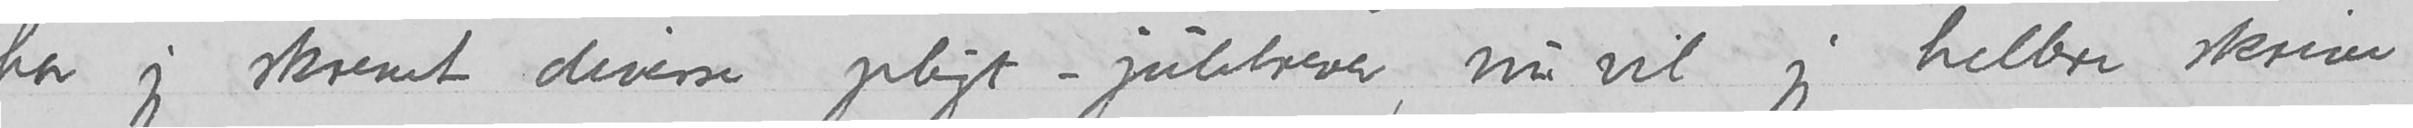har jeg skrevet diverse pligt-julebrever, nu vil jeg hellere skrive
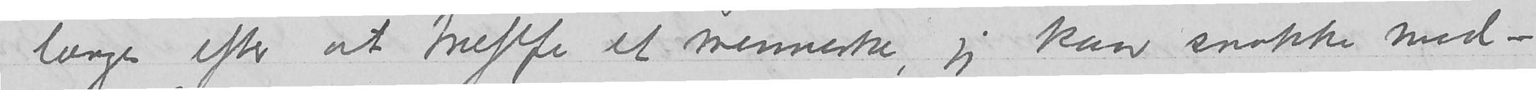Jeg længes efter at træffe et menneske, jeg kan snakke med –
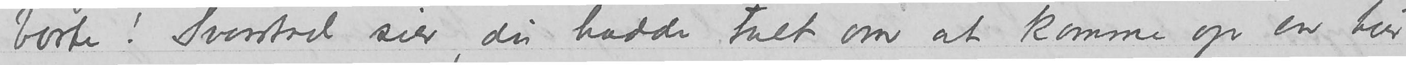borte! Svarstad sier, du hadde talt om at komme op en tur
